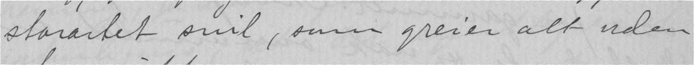storartet snil, som greier alt uden
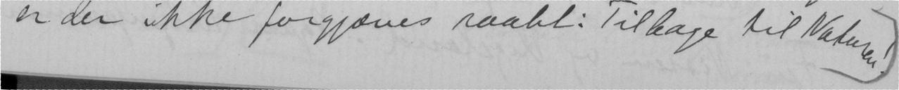er der ikke forgjæves raabt: Tilbage til Naturen!
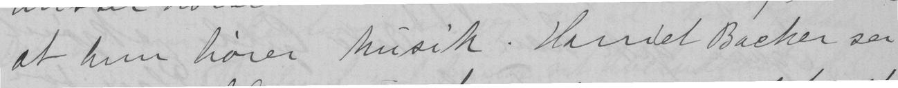at hun hører Musik. Harriet Backer ser
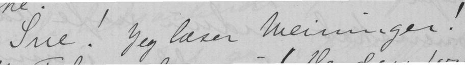Sne! Jeg læser Weininger!
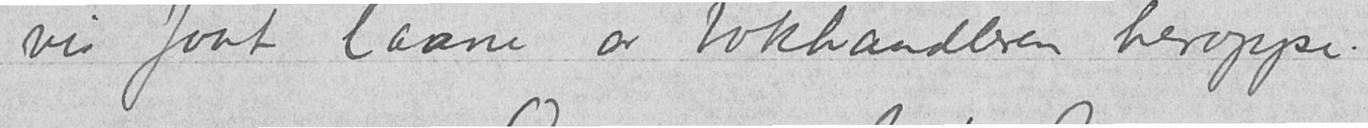vis faat laane av bokhandleren heroppe.
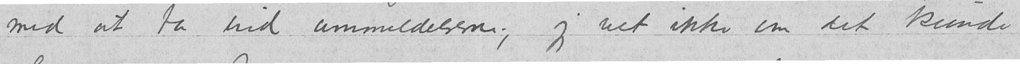med at ta ind ammeldelserne; jeg vet ikke om det kunde
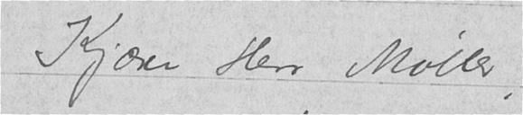Kjære Herr Møller
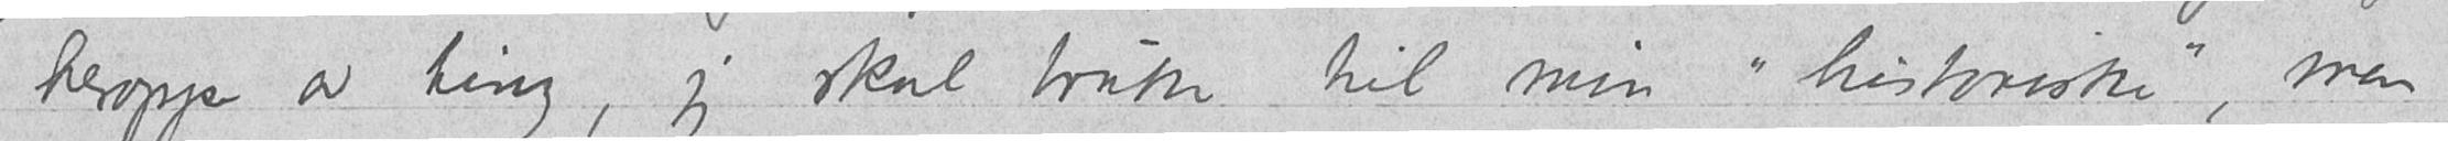heroppe av ting, jeg skal bruke til min "historiske", men
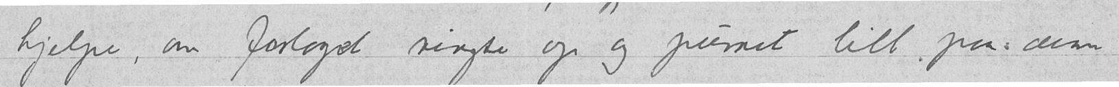hjelpe, om forlaget ringte op og purret litt paa dem
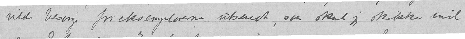vilde besørge frieksemplarerne utsendt, saa skal jeg skikke ind
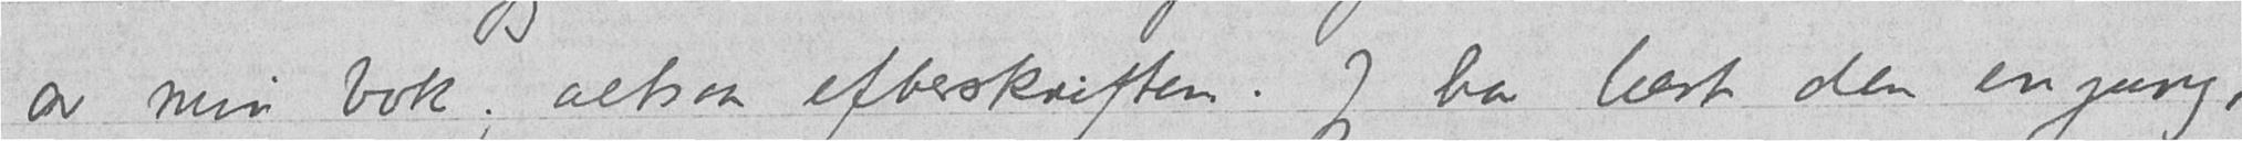av min bok; altsaa efterskriften. Jeg har læst den engang,
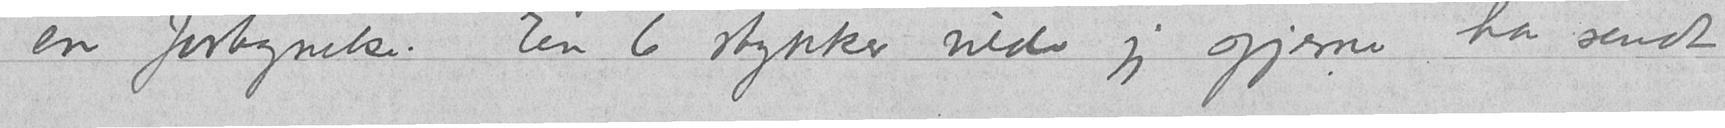en fortegnelse. En 6 stykker vilde jeg gjerne ha sendt
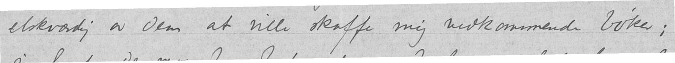elskværdig av Dem at ville skaffe mig vedkommende bøker;
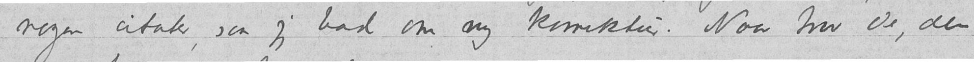nogen citater, saa jeg bad om ny korrektur. Naar tror De, den
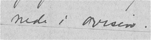nede i avisen.
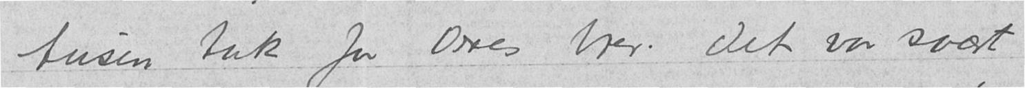tusen tak for Deres brev. Det var svært
